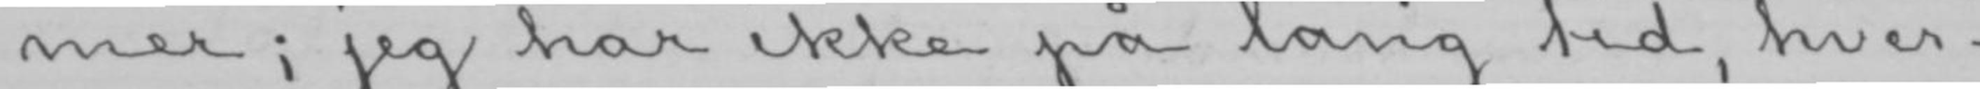mer; jeg har ikke på lang tid, hver-
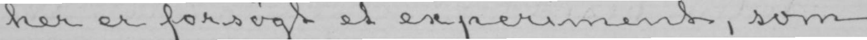her er forsøgt et experiment, som
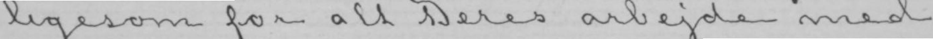ligesom for alt Deres arbejde med
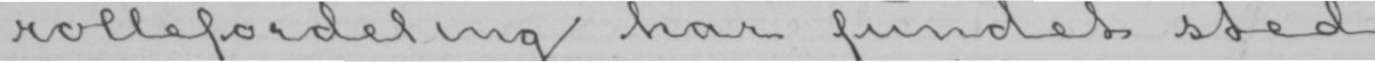rollefordeling har fundet sted
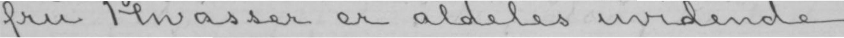fru Hwasser er aldeles uvidende
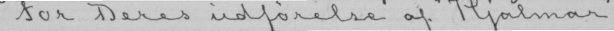For Deres udførelse af «Hjalmar»
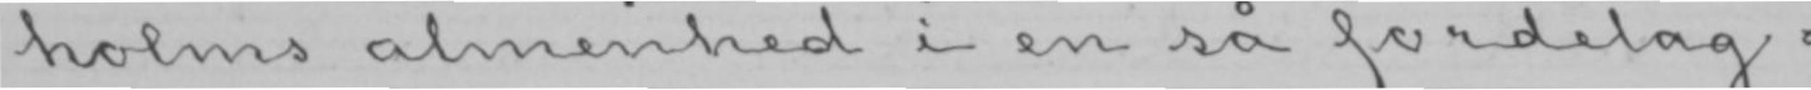holms almenhed i en så fordelag-
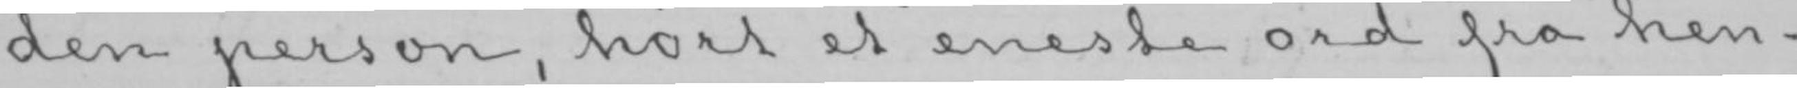den person, hørt et eneste ord fra hen-
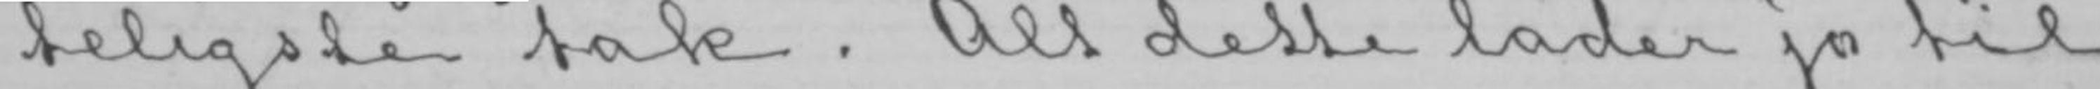teligste tak. Alt dette lader jo til
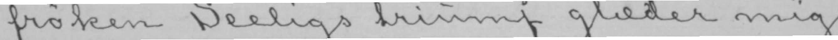frøken Seeligs triumf glæder mig
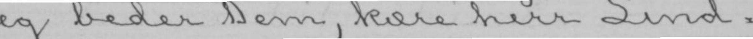Jeg beder Dem, kære herr Lind-
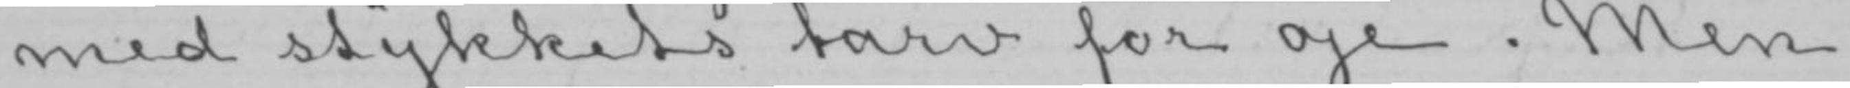med stykkets tarv for øje. Men
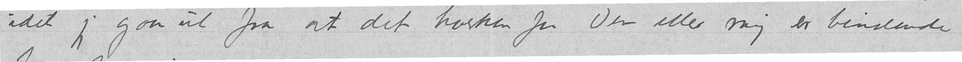idet jeg gaar ut fra at det hverken for Dem eller mig er bindende
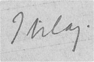1 bilag.
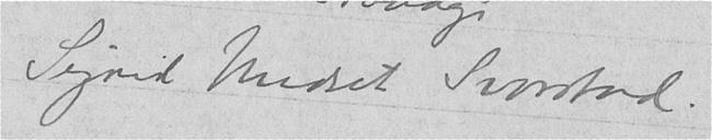Sigrid Undset Svarstad.
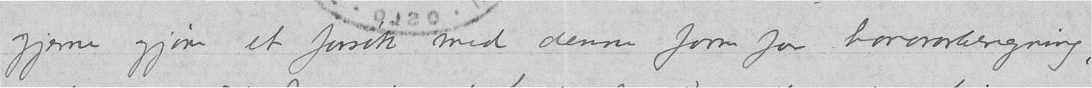gjerne gjøre et forsøk med denne form for honorarberegning,
